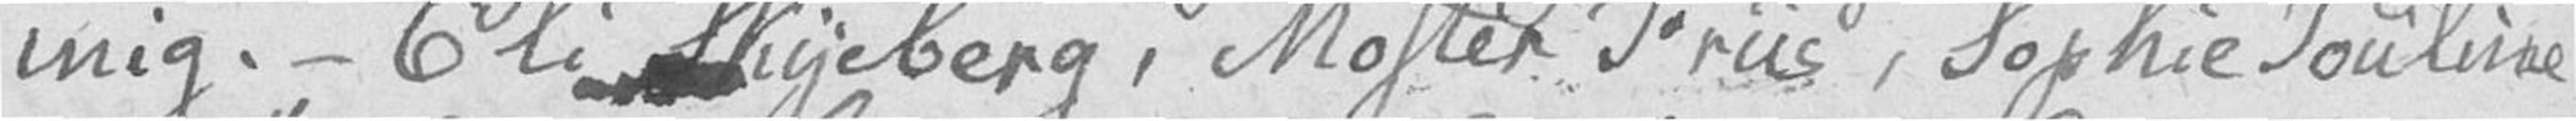mig. – Eli Skyeberg, Moster Friis, Sophie Pouline
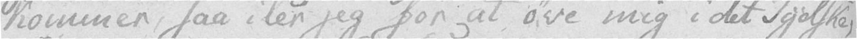kommer, saa iler jeg for at øve mig i det Tydske,
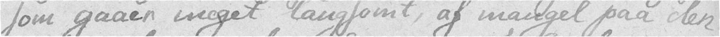som gaaer meget langsomt, af mangel paa den
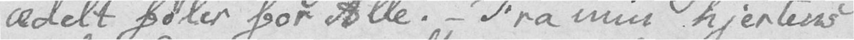ædelt føler for Alle. – Fra min hjertens
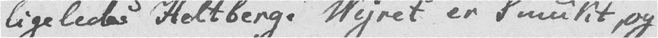ligeledes Heltberg. Vejret er Smukt, og
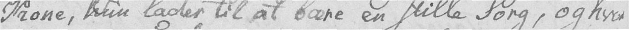Kone, hun lader til at bære en stille Sorg, og hva
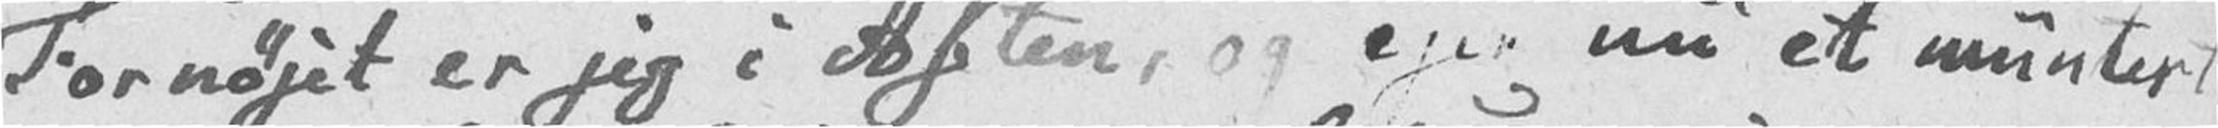Fornøjet er jeg i Aften, og ejer nu et muntert
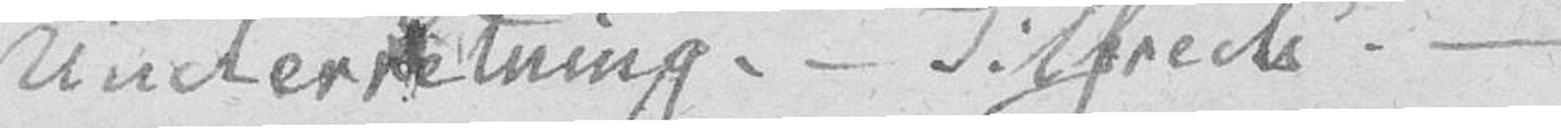Underretning. – Tilfreds. –
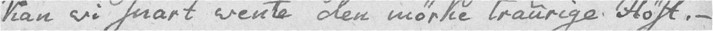kan vi snart vente den mørke traurige Høst. –
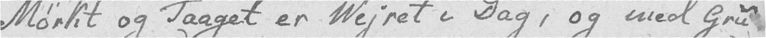Mørkt og Taaget er Vejret i Dag, og med Gru
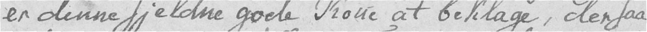er denne sjeldne gode Kone at beklage, der saa
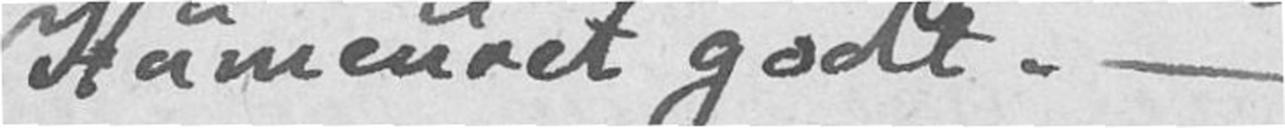Humeuret godt. –
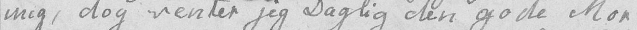mig, dog venter jeg Daglig den gode Mor
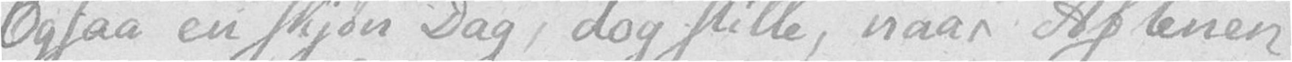Ogsaa en skjøn Dag, dog stille, naar Aftenen
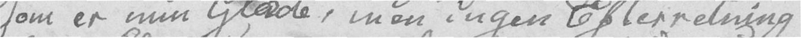som er min Glæde, men ingen Efterretning
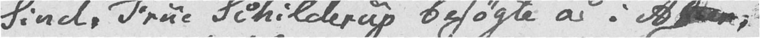Sind. Frue Schilderup besøgte os i Aften,
